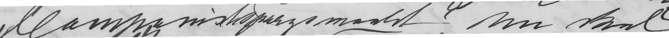Camponistspørgsmaalet. Nu skal
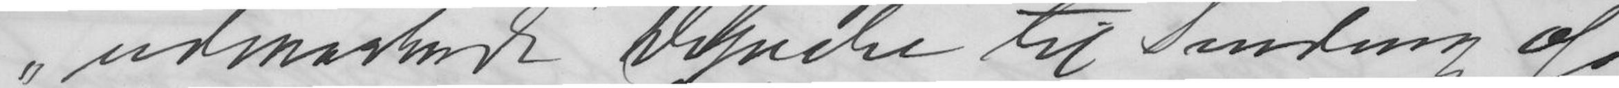udmærkede Depeche til Sinding osv.
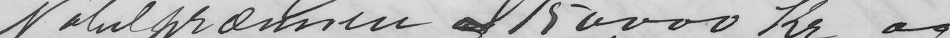Nobelpræmien og 150.000 Kr og
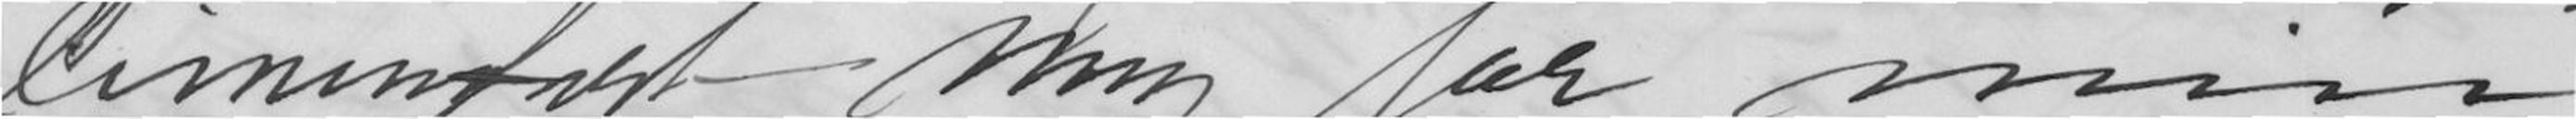limenteret mig for min
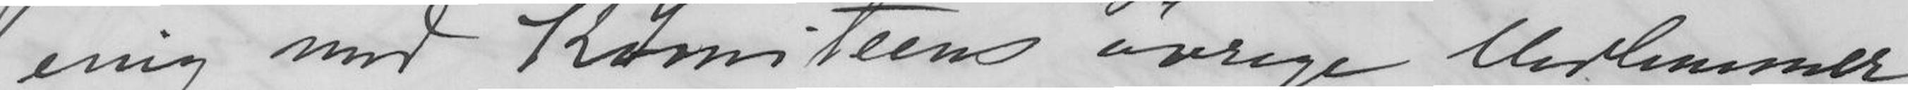enig med Komiteens øvrige Medlemmer
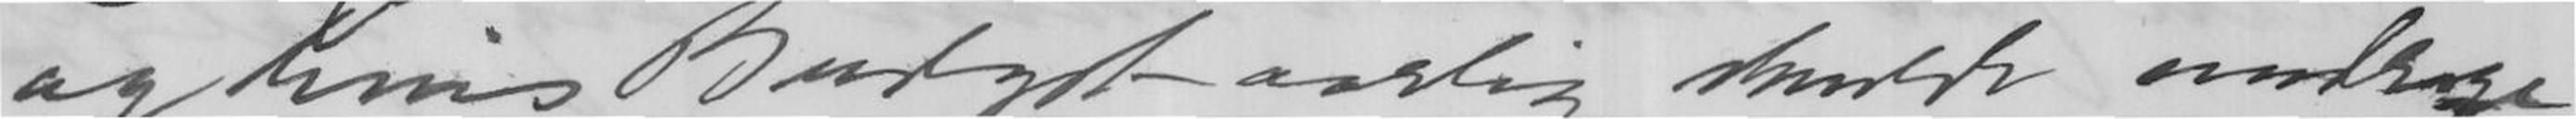og hvis Budget aarlig skulde undrage
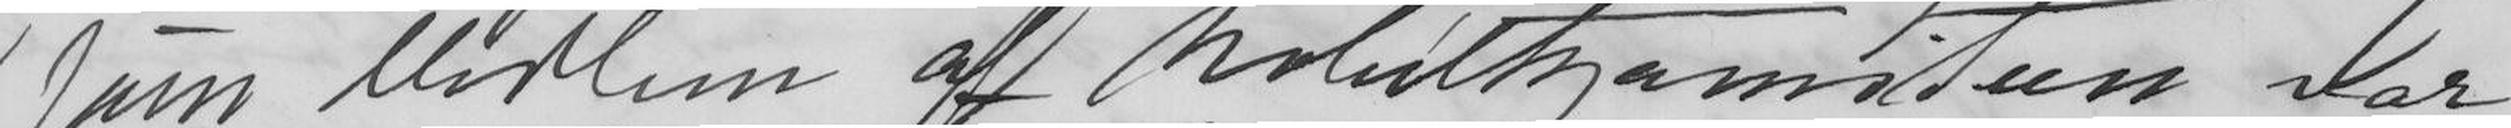som Medlem af Nobelkomiten var
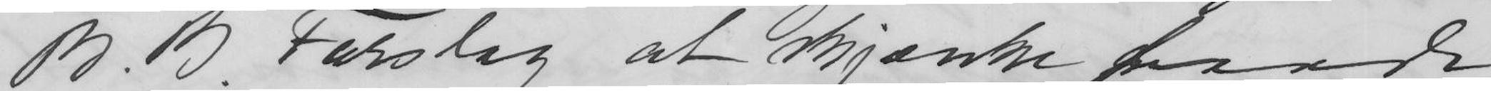B. B. Forslag at skjænke baade
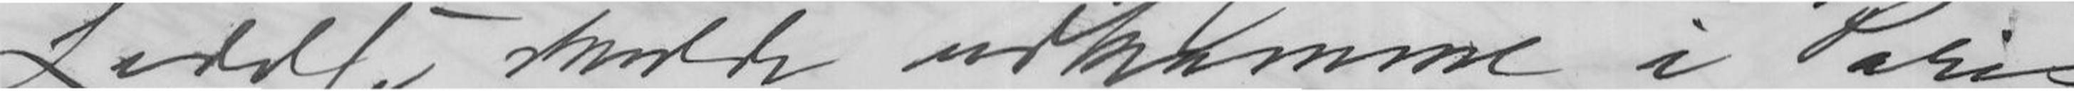Ledelse skulde udkomme i Paris
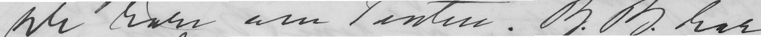De høre om Texten. B. B. har
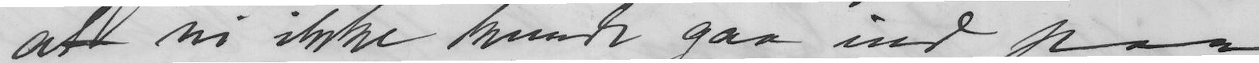at vi ikke kunde gaa ind paa
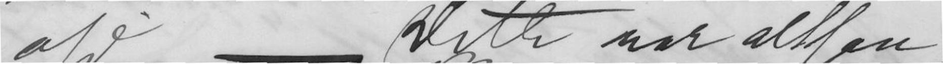osv. - Dette var altsaa
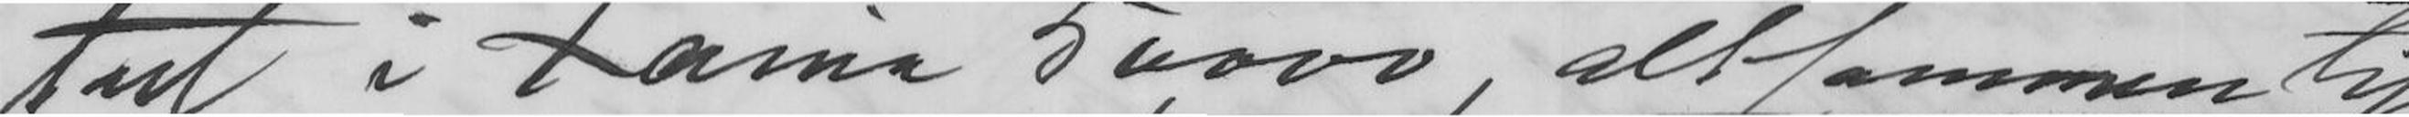tut i Xania 50.000, altsammen til
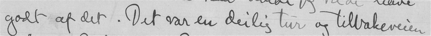godt af det. Det var en deilig tur og tilbakeveien
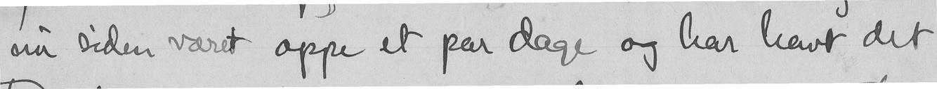nu siden været oppe et par dage og har havt det
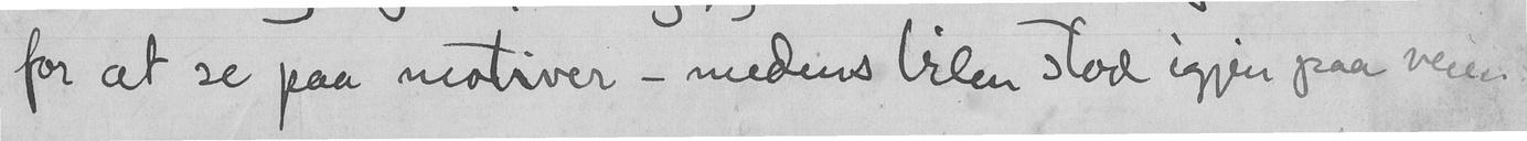for at se paa motiver - medens bilen stod igjen paa veien.
